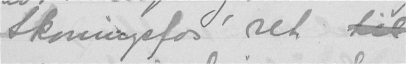Skoningsfos ret til
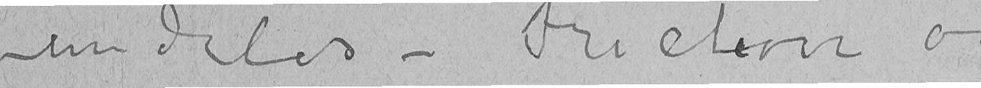fremdeles – Friction og
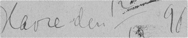Havre den 12/12 90
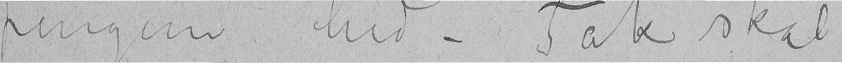pengene hid – Tak skal
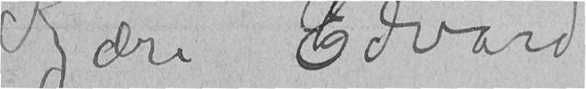Kjære Edvard
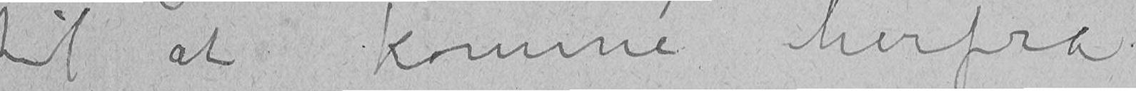til at komme herfra
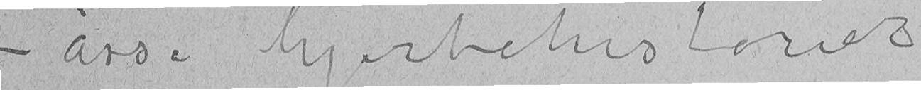– åsså hjertehistorier –
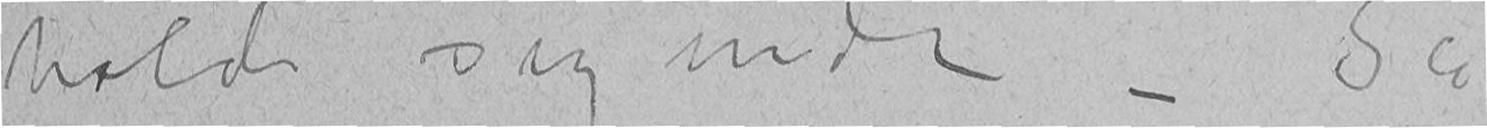holde sig inde – Så
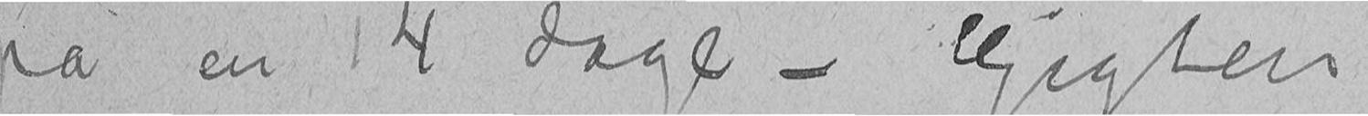på en 14 dage – Gigten
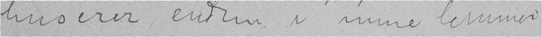huserer endnu i mine lemmer
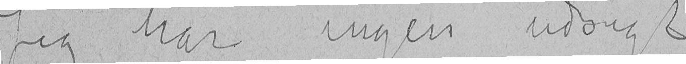Jeg har ingen udsigt
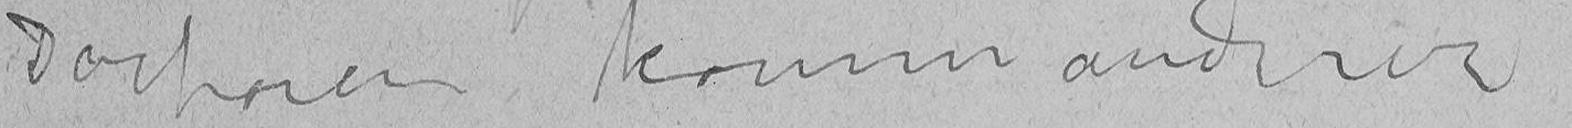Doctoren kommanderer
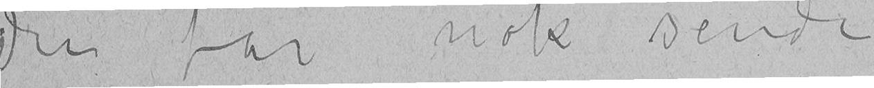Du får nok sende
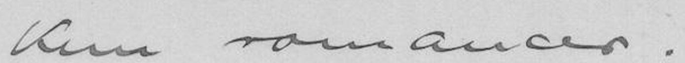kun romancer.
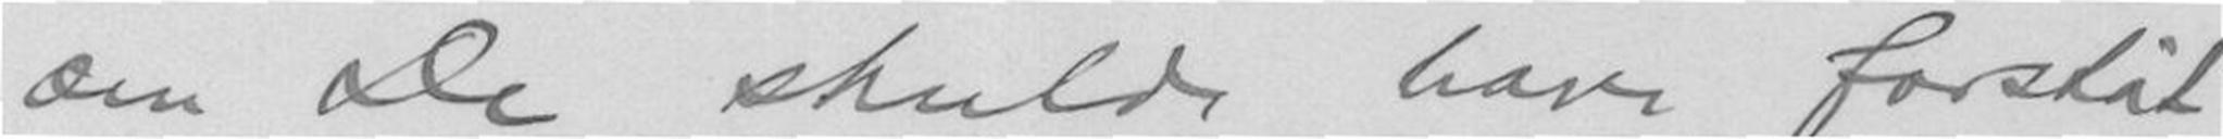om De skulde have forståt
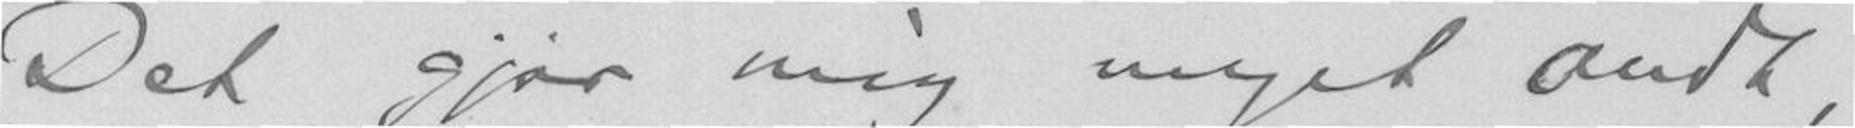Det gjør mig meget ondt,
